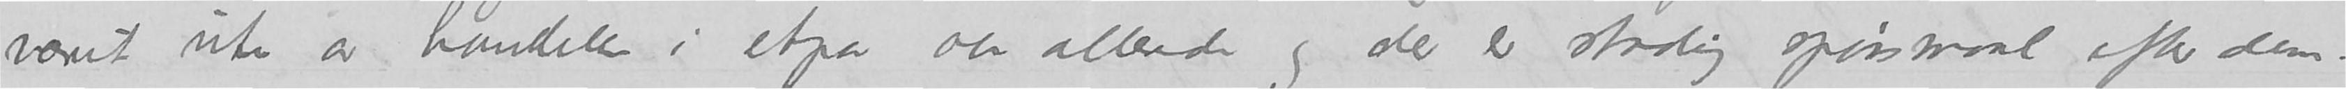været ute av handelen i etpar aar allerede og der er stadig spørsmål efter dem.
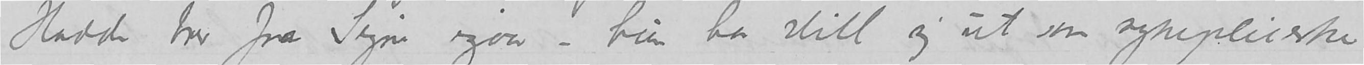Hadde brev fra Signe igaar – hun har slitt seg ut som sykepleierske
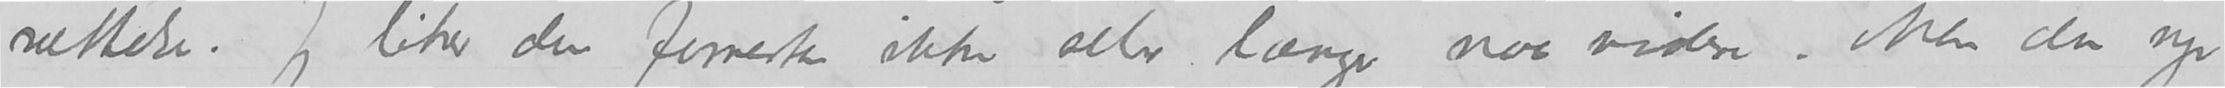sættelse. Jeg liker den forresten ikke selv længer noe videre. Men den nye
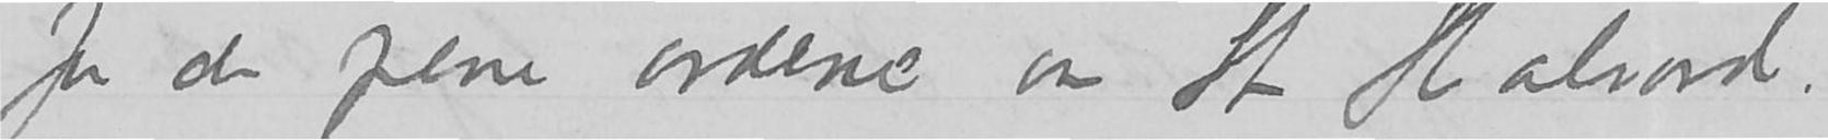for de pene ordene om St. Halvard.
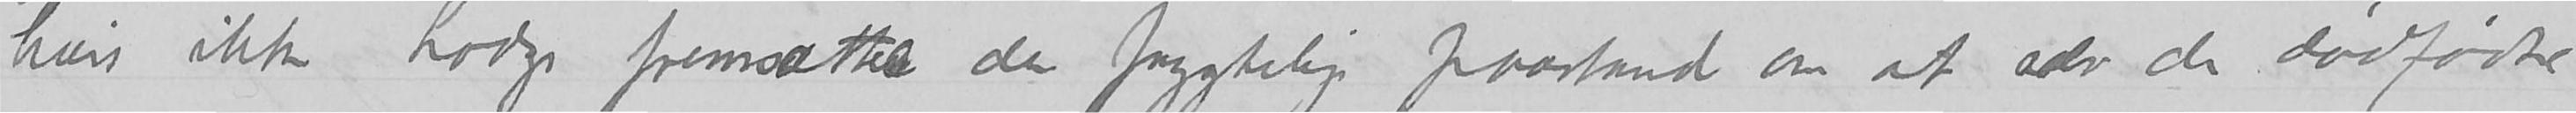hvis ikke Lodge fremsætter den frygtelige paastand om at alle selv de dødfødte
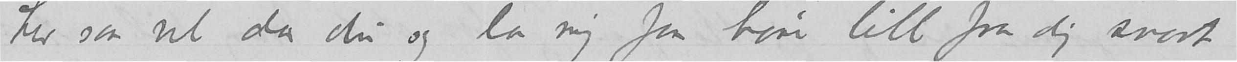Lev saa vel da du og la mig faa høre litt fra dig snart –
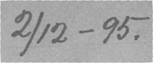2/12-95.
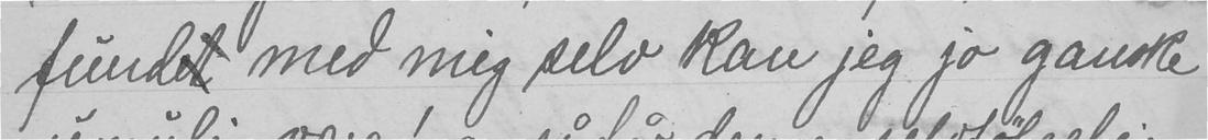fundet med meg selv kan jeg jo ganske
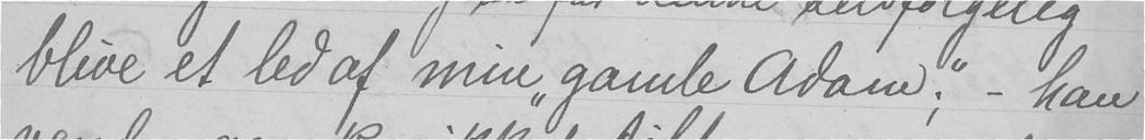blive et led af min "gamle Adam;" - han
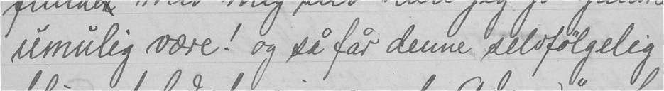umulig være! og så får denne selvfølgelig
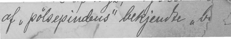af "pølsepindens" bekjendte
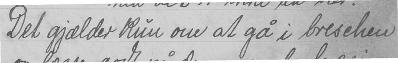Det gjælder kun om at gå i breschen
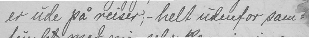er ude på reiser, - helt udenfor sam-
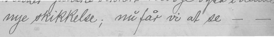nye skikkelse; nu får vi at se - -
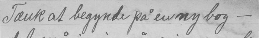Tænk at begynde på en ny bog -
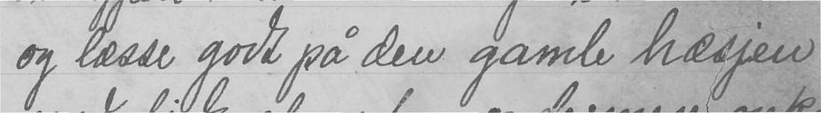og læsse godt på den gamle hæsjen
